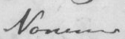Nonnerne.
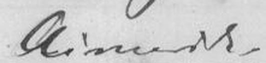Aimerik.
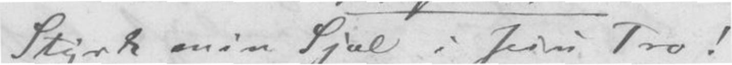Styrk min Sjæl i Jesu Tro!
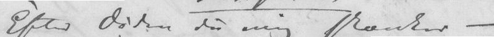Efter Døden du mig skænker -
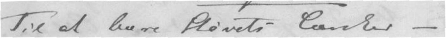Tid at have Støvets Lænker -
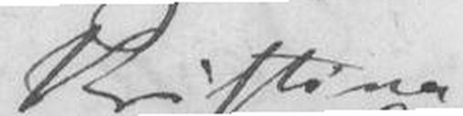Kristina.
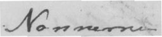Nonnerne
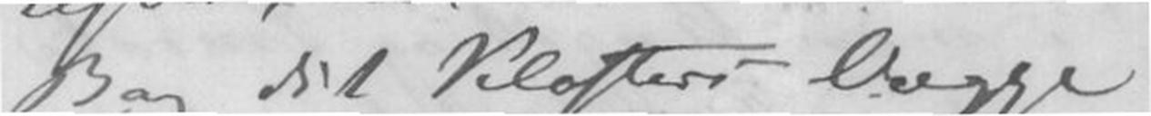Bag dit Klosters Vægge
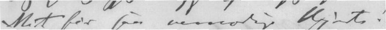Mit før saa vemodige Hjerte!
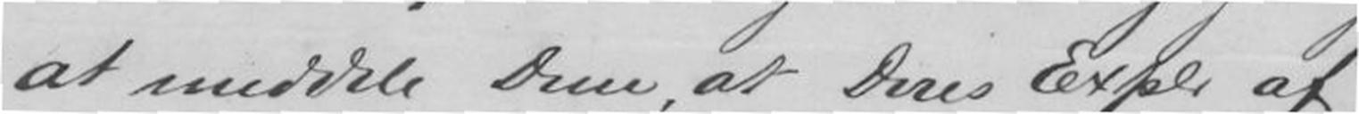at meddele Dem, at Deres Expl. af
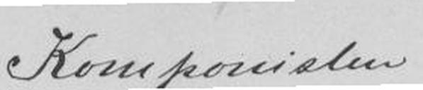Komponisten
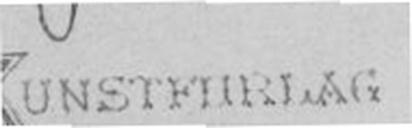KUNSTFORLAG
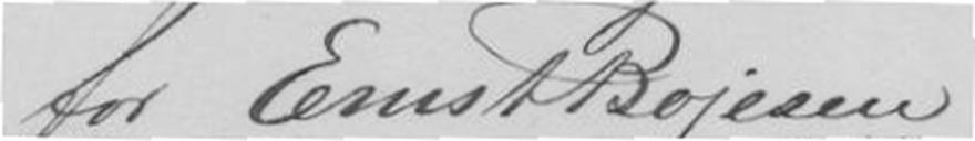for Ernst Bojesen
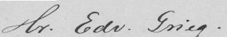Hr. Edv. Grieg!
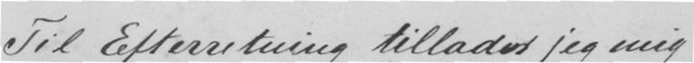Til Efterretning tillader jeg mig
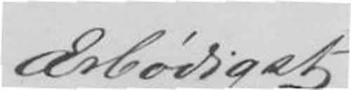Erbødigst
